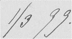1/3 99.
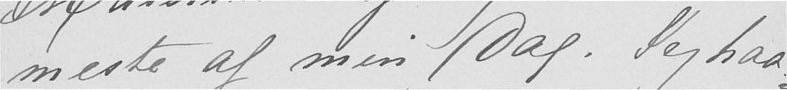meste af min Dag. Jeg haa-
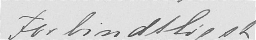Forbindtligst
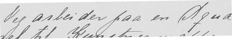Jeg arbeider paa en Aqua-
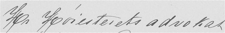Hr Høiesteretsadvokat
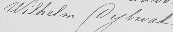Wilhelm Dybwad
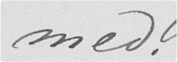med.
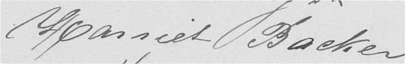Harriet Backer
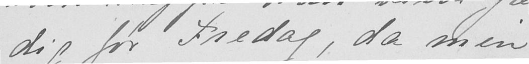dig før Fredag, da min
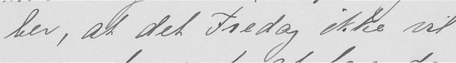ber, at det Fredag ikke vil
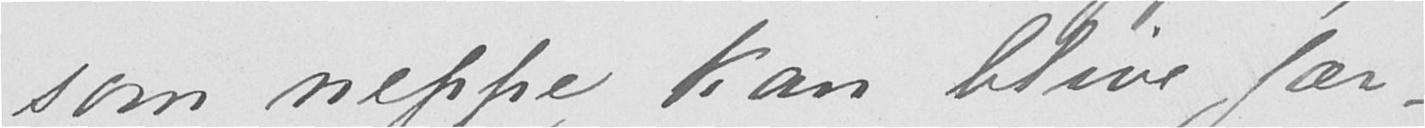som neppe kan blive fær-
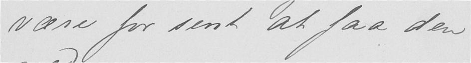være for sent at faa den
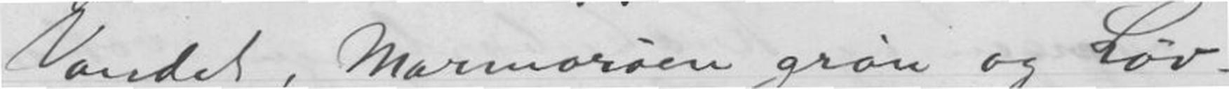Vandet, Marmorøen grøn og Løv-
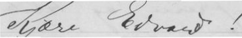Kjære Edvard!
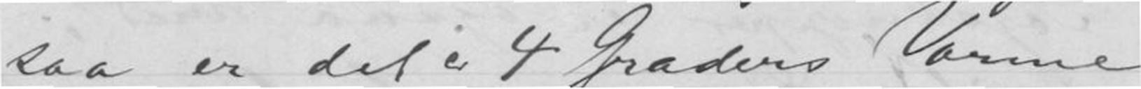saa er det ca 4 Graders Varme,
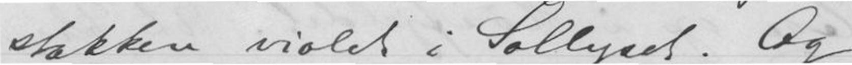stakken violet i Sollyset. Og
